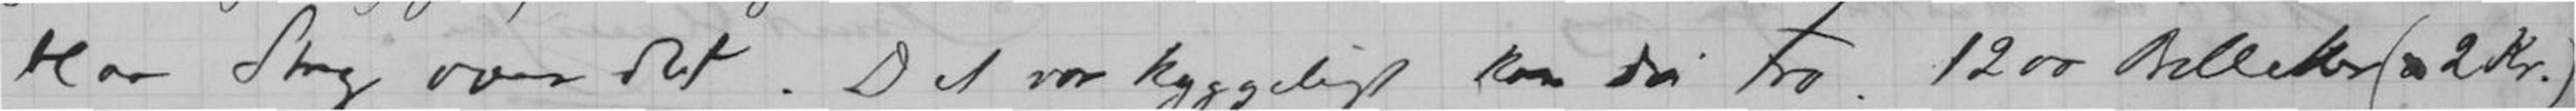blaa Streg over det. Det var hyggeligt kan Du tro. 1200 Billetter (a 2 Kr.)
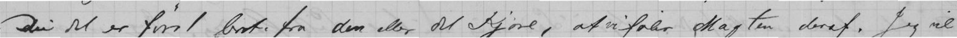Du det er først borte fra den eller det Kjære, at vi føler Magten deraf. Jeg vil
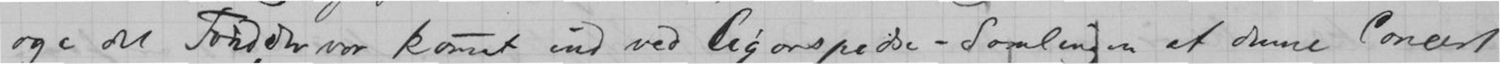og i det Fond der var komet ind ved Cigarspedse-Samlingen at denne Concert
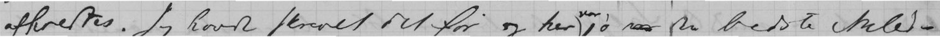afhørtes. Jeg havde skrevet det for og her var jo den bedste Anled-
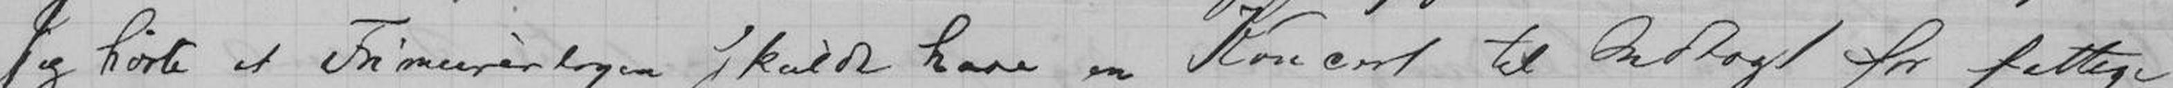Jeg hørte at Frimurerlogen skulde have en Koncert til Indtægt for fattige
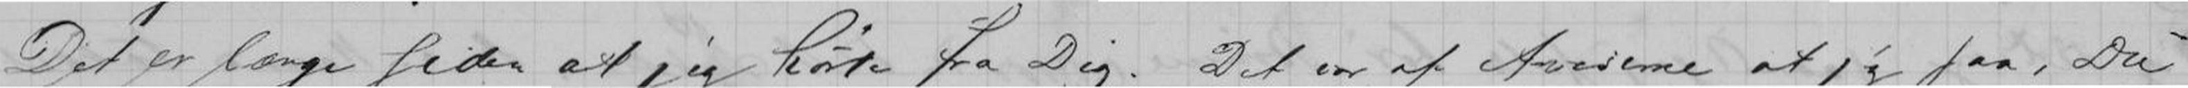Det er længe siden at jeg hørte fra Dig. Det var af Aviserne at jeg saa, Du
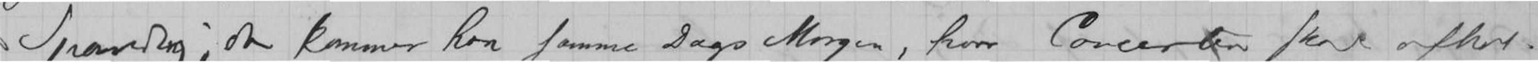Spændig; der kommer han samme Dags Morgen, hvor Concerten skal afhol-
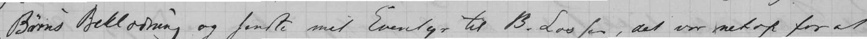Børns Beklædning og sendte mit Eventyr til B. Losser, det var netop for at
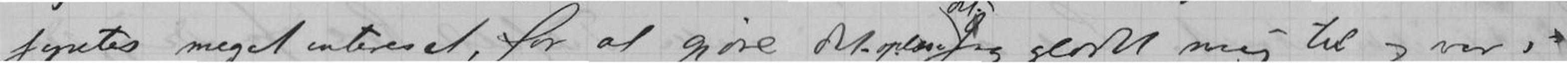syntes meget intereset, for at gjøre det, opløse det. Jeg glædet mig til, vor,
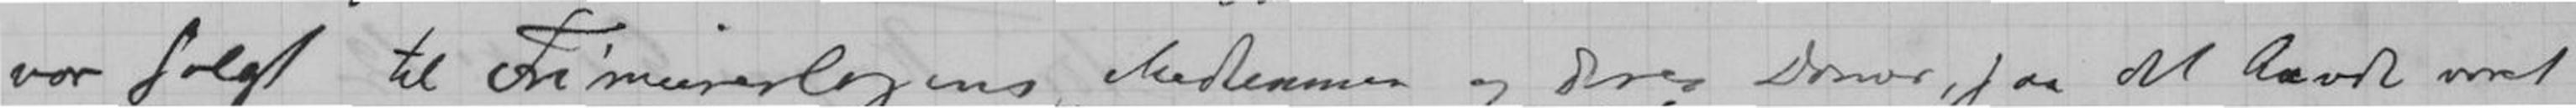var solgt til Frimurerlogens Medlemmer og Deres Damer, saa det havde været
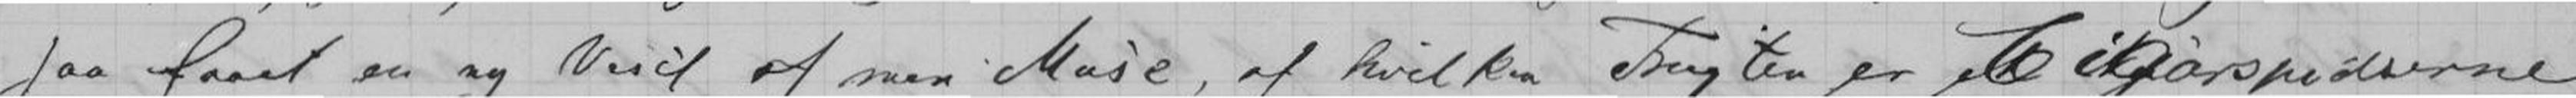saa faaet en ny Visit af min Muse, af hvilken Frygten er Cigarspidserne
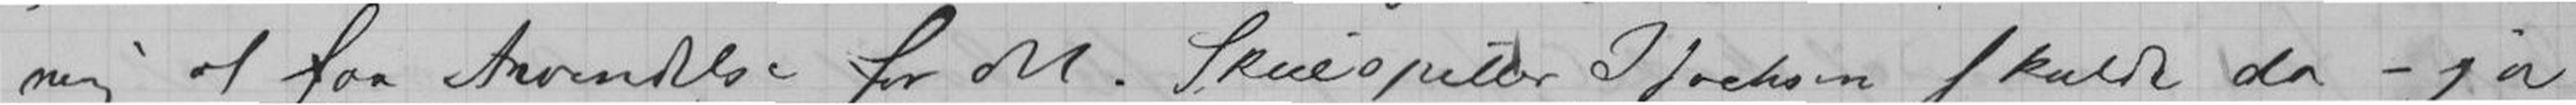ning af faa Anvendelse for det. Skuespiller Isachsen skulde da - ja
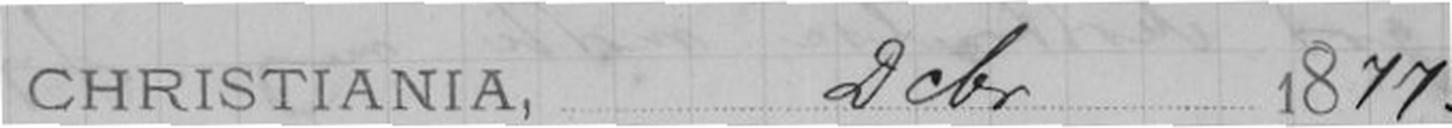Christiania, Dcbr. 1877
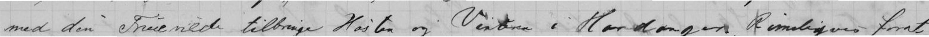med din Frue vilde tilbringe Høsten og Vinteren i Hardanger. Rimeligvis forat
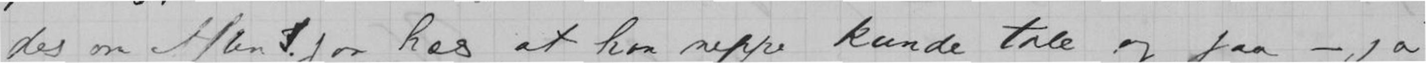des om Aften. Ssaa hæs at han neppe kunde tale og saa - ja
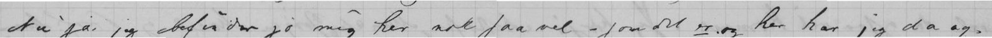Nu ja jeg befinder jo mig her nok saa vel - som det er og her har jeg da og-
